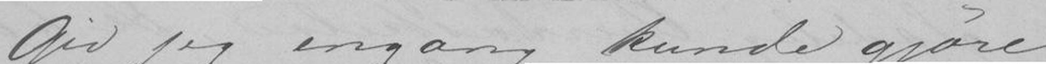Gid jeg engang kunde gjøre
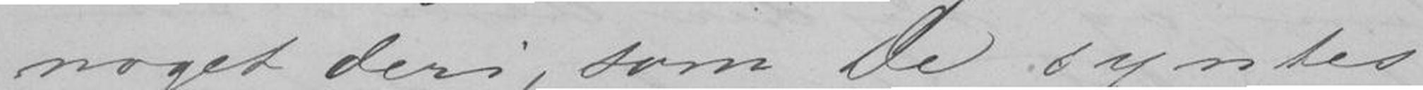noget deri, som De syntes
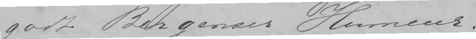godt Bergenser Humeur;
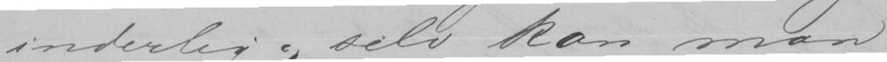inderlig; selv kan man
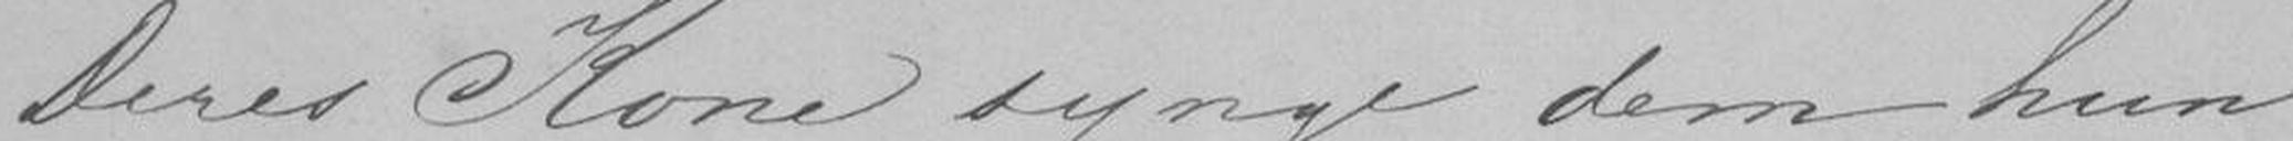Deres Kone synge dem, hun
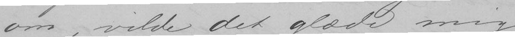om, vilde det glæde mig
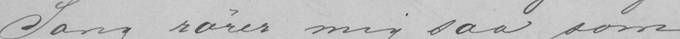Sang rører mig saa som
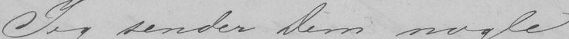Jeg sender Dem nogle
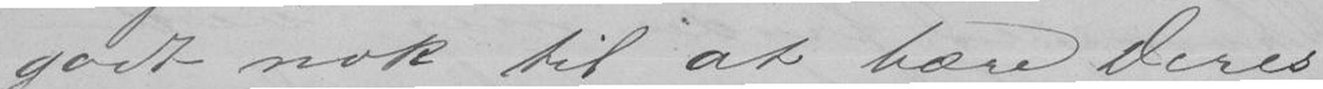godt nok til at bære Deres
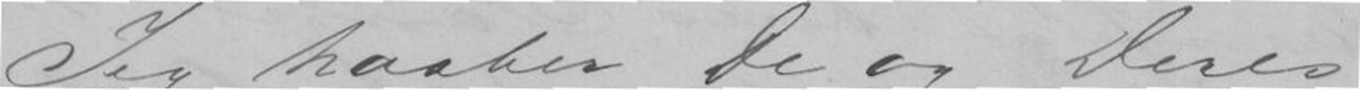Jeg haaber De og Deres
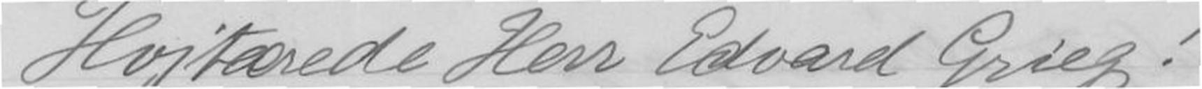Højtærede Herr Edvard Grieg!
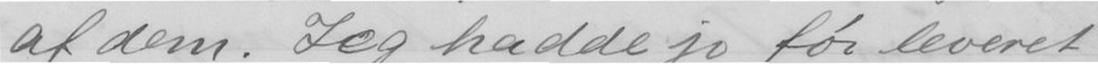af dem. Jeg hadde jo før leveret
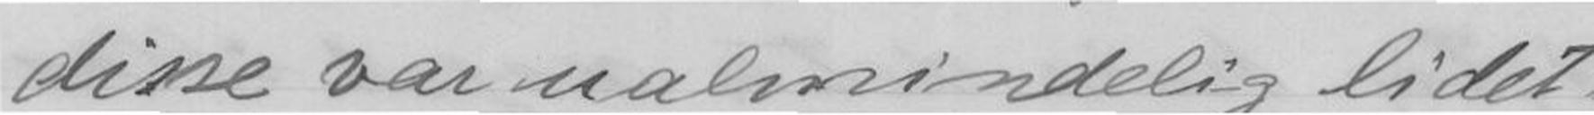disse var ualmindelig lidet
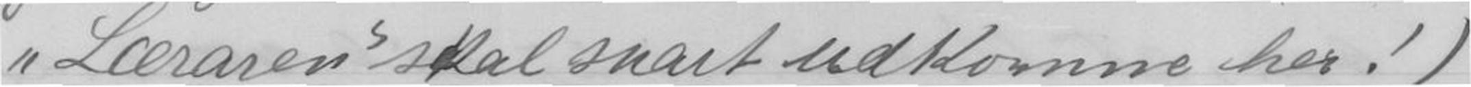"Læraren" skal snart udkomme her!)
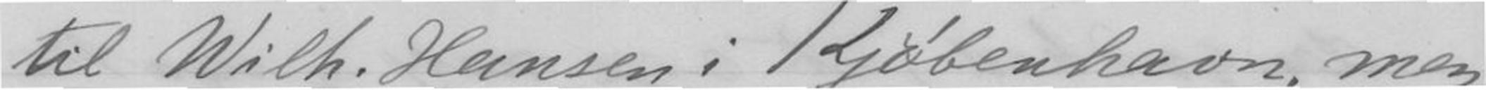til Wilh. Hansen i Kjøbenhavn, men
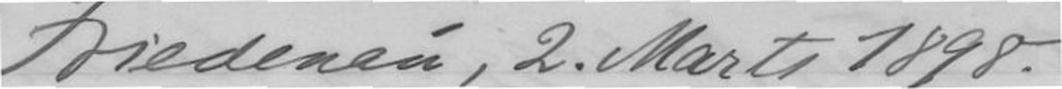Friedenau, 2. Marts 1898.
